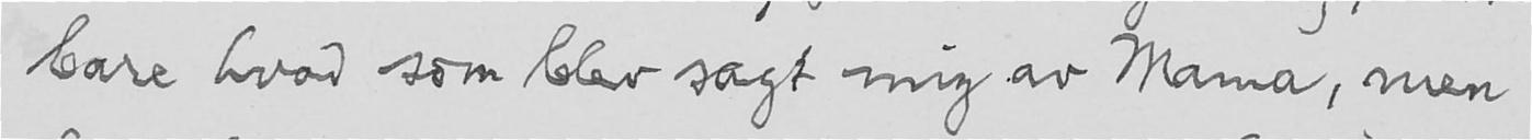bare hvad som blev sagt mig av Mama, men
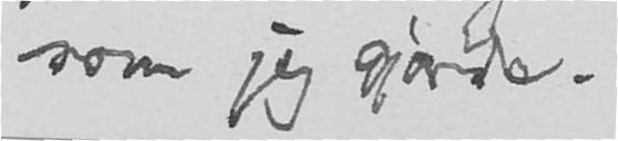som jeg gjorde.
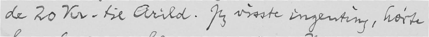de 20 Kr. til Arild. Jeg visste ingenting, hørte
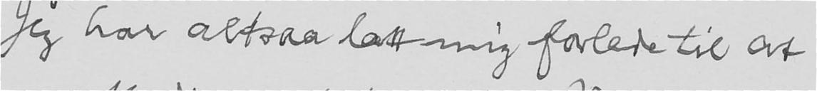Jeg har altsaa latt mig forlede til at
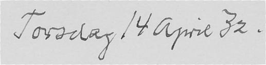Torsdag 14 April 32.
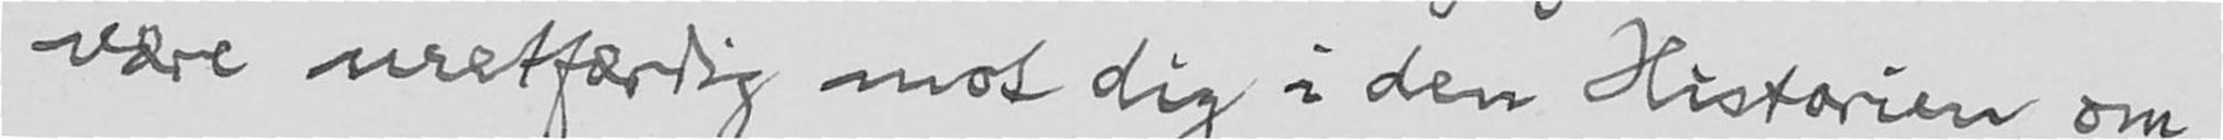være uretfærdig mot dig i den Historien om
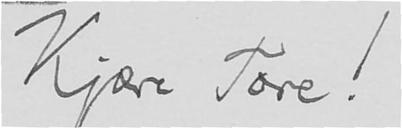Kjære Tore!
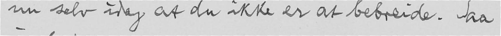nu selv idag at du ikke er at bebreide. Saa
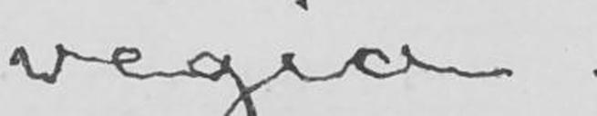vegia.
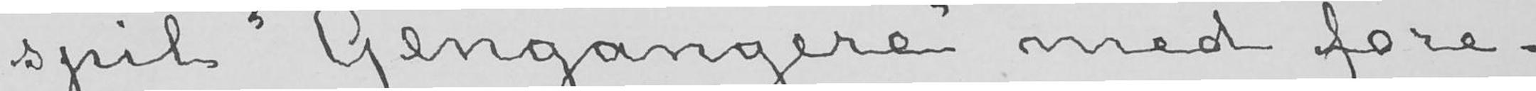spil «Gengangere» med fore-
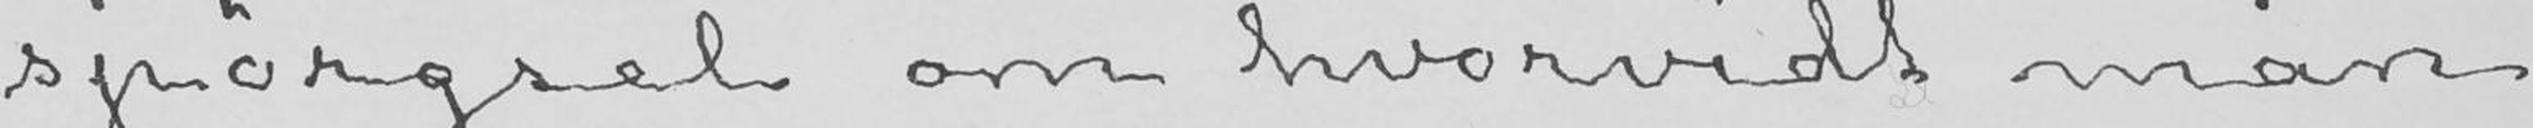spørgsel om hvorvidt man
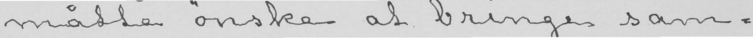måtte ønske at bringe sam-
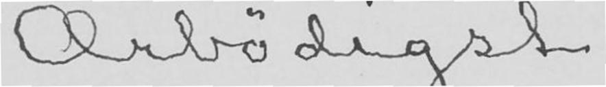Ærbødigst
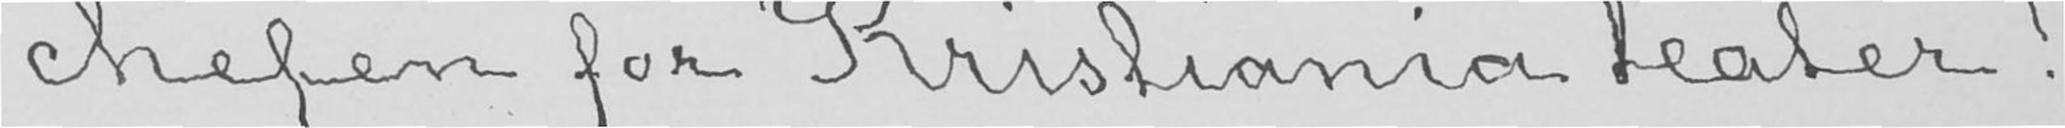chefen for Kristiania teater!
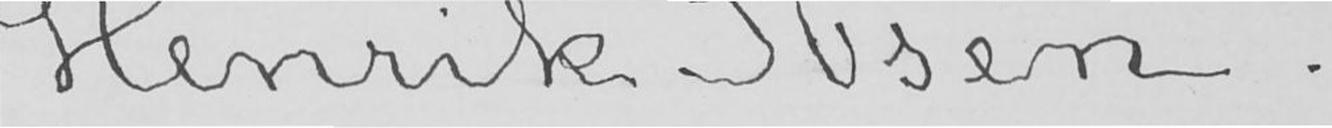Henrik Ibsen.
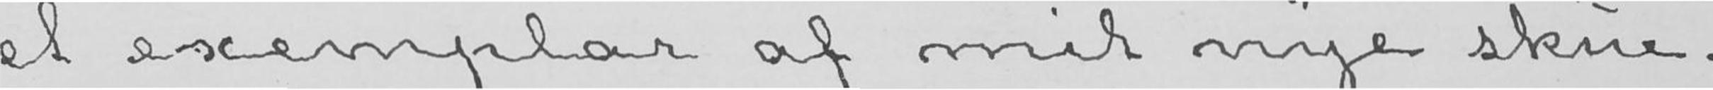et exemplar af mit nye skue-
